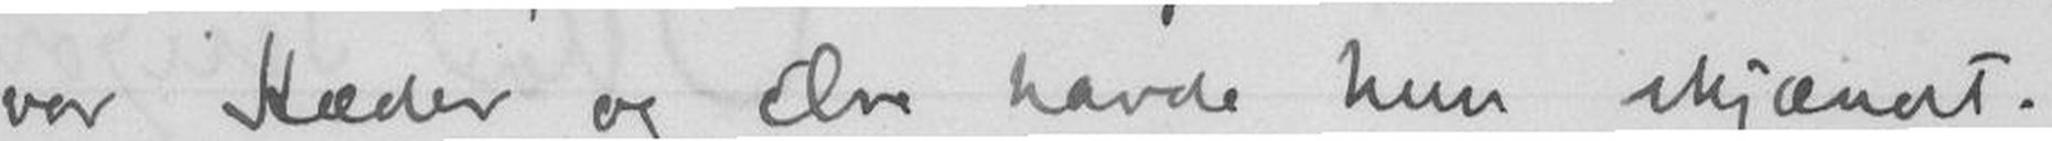vor Hæder og Ære havde hun skjæmdt.
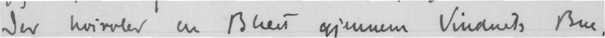Der hvirvler en Blæst gjennem Vinduets Bue.
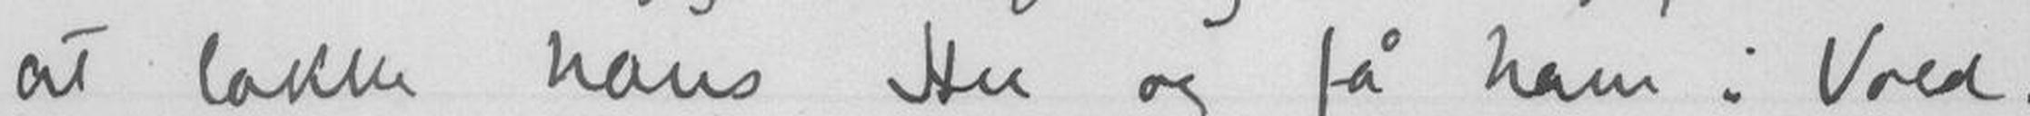at lokke hans Hu og få ham i Vold.
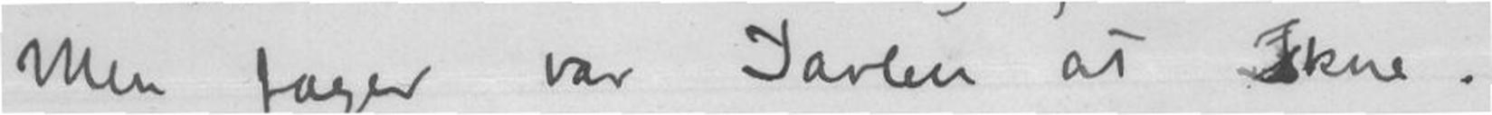Men fager var Jarlen at skue.
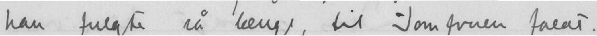han fulgte så længe, til Jomfruen faldt.
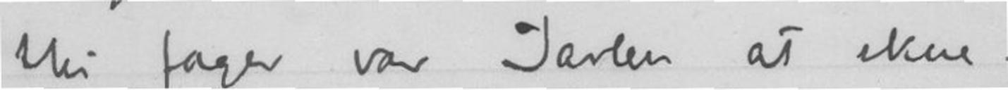thi fager var Jarlen at skue.
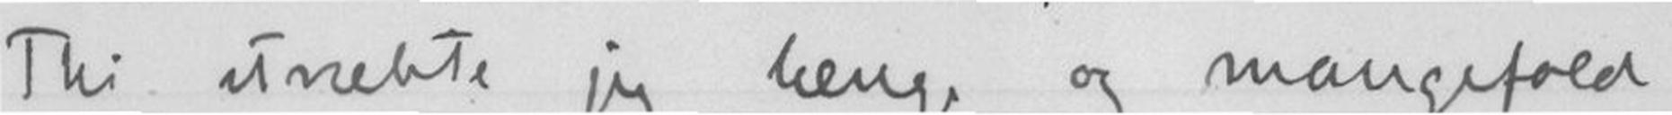Thi stræbte jeg længe og mangefold
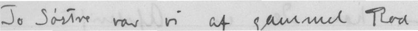To Søstre var vi af gammel Rod,
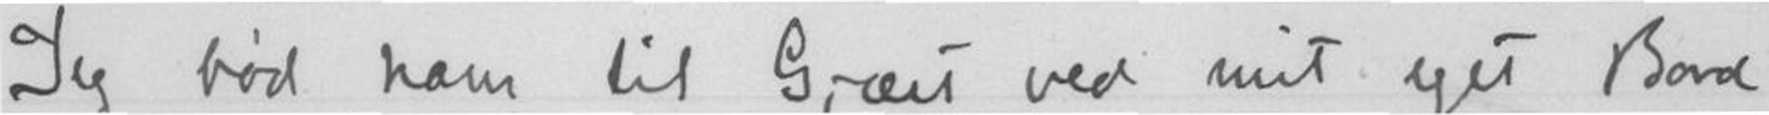Jeg bød ham til Gjæst ved mit eget Bord,
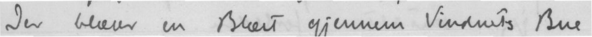Der blæser en Blæst gjennem Vinduets Bue.
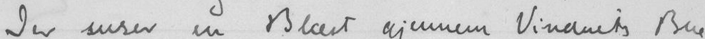Der suser en Blæst gjennem Vinduets Bue.
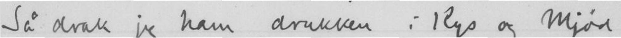Så drak jeg ham drukken i Kys og Mjød
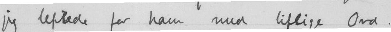jeg leftede for ham med liflige Ord.
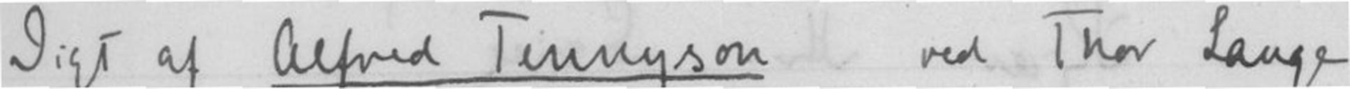Digt af Alfred Tinnysonved Thor Lange
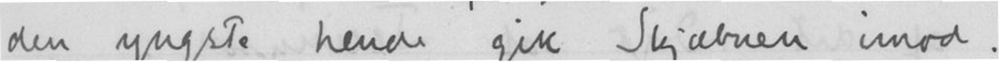den yngste hende gik Skjebnen imod.
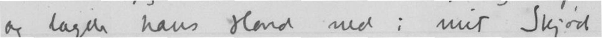og lagde hans Hand ned i mit Skjød,
